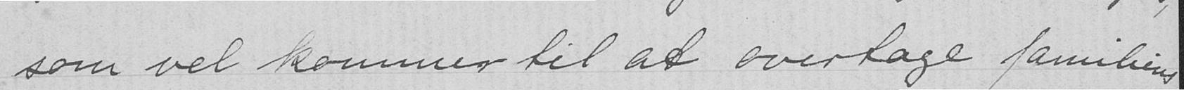som vel kommer til at overtage familiens
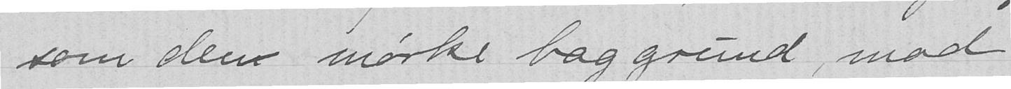som den mørke baggrund, mod
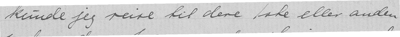kunde jeg reise til dere 1ste eller anden
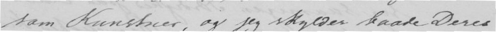som Kunstner, og jeg skylder baade Deres
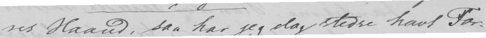res Haand, saa har jeg dog stedse havt For-
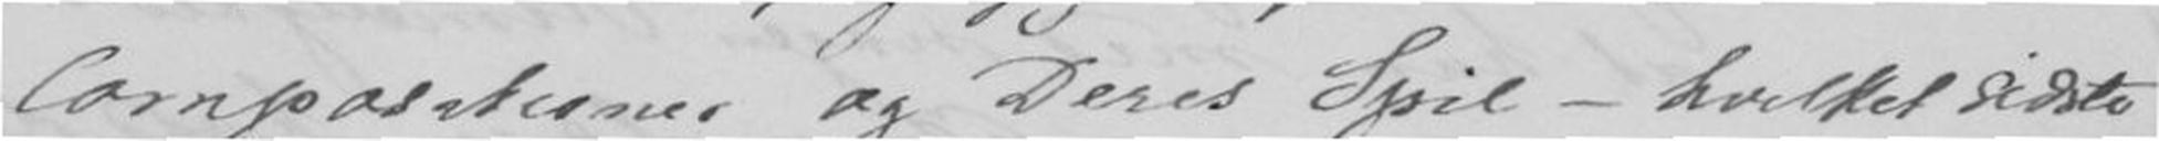Compositioner og Deres Spil - hvilket sidste
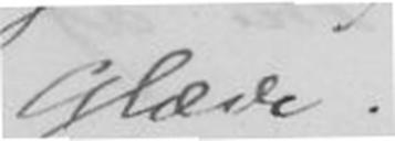Glæde.
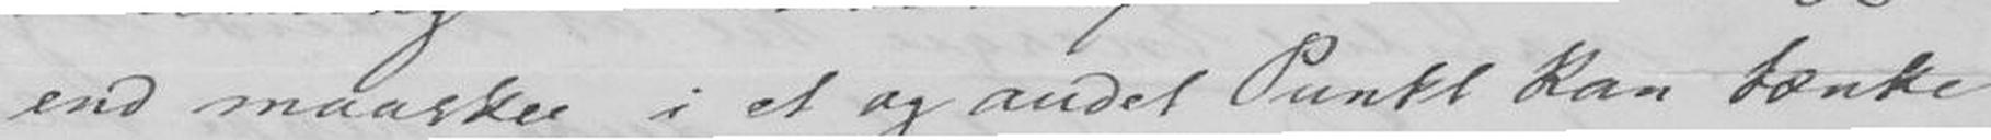end maaskee i et og andet Punkt kan tænke
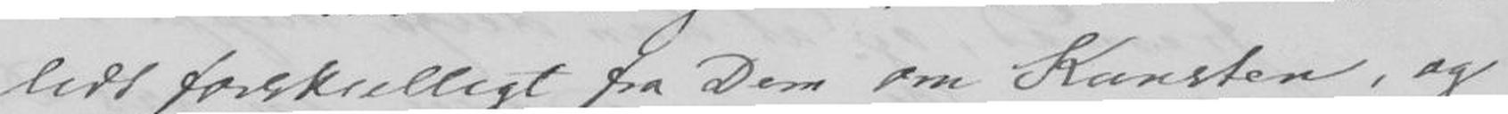lidt forskelligt fra Dem om Kunsten, og
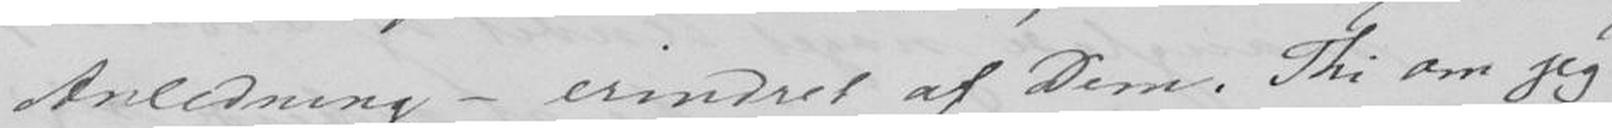Anledning - erindret af Dem. Thi om jeg
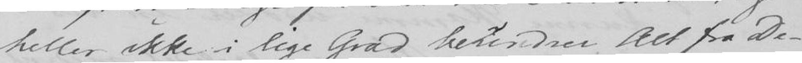heller ikke i lige Grad beundrer alt fra De-
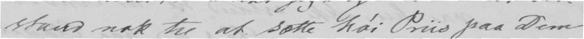stand nok til at sætte høi Pris paa Dem
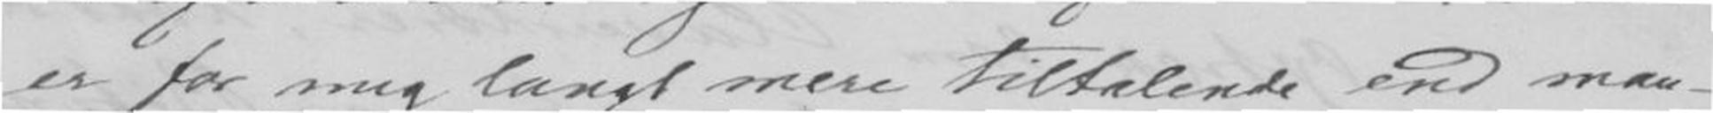er for mig langt mere tiltalende end man-
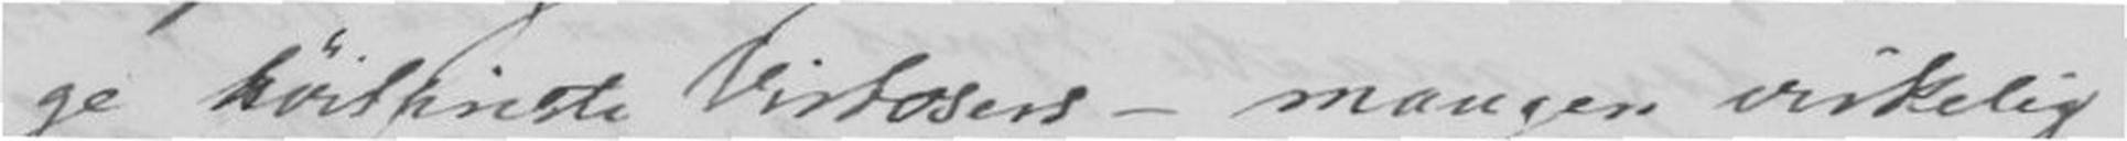ge høithviste Virtosers - mangen rigelig
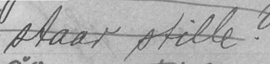staar stille?
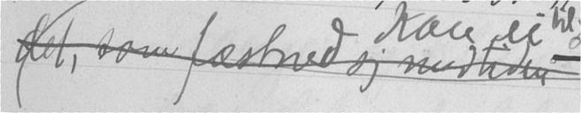det, som fæstned sj med tiden
